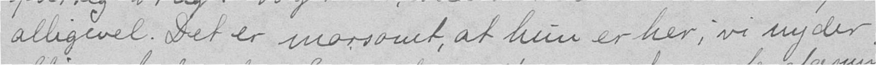alligevel. Det er morsomt, at hun er her; vi nyder
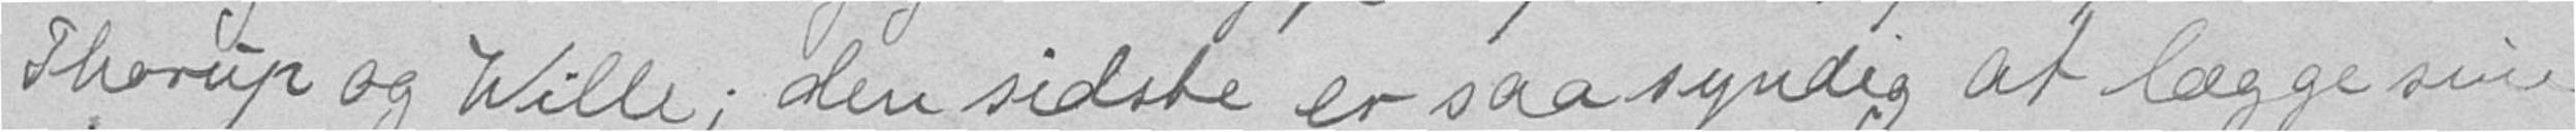Thorup og Wille; den sidste er saa syndig at lægge sine
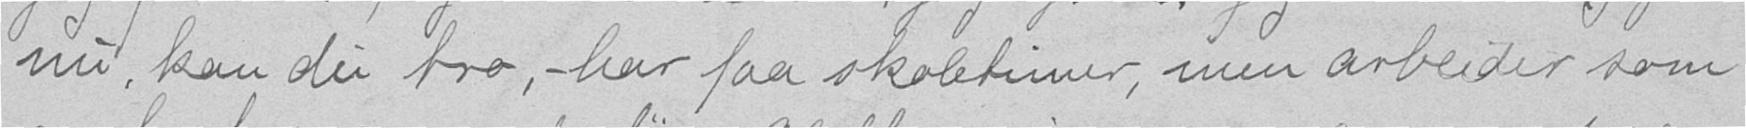nu, kan de tro, - har faa skoletimer, men arbeider som
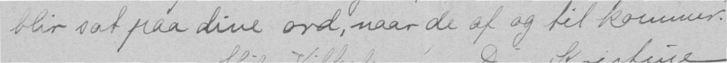blir sat paa dine ord, naar de af og til kommer.
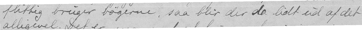flittig bruger bøgerne, saa blir der da lidt ud af det
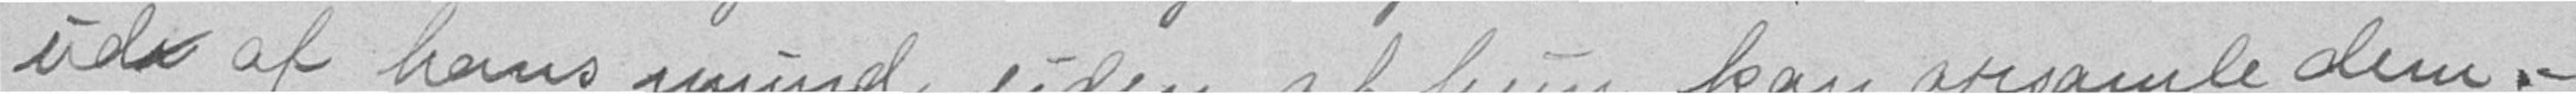ud af hans mund, uden at hun kan opsamle dem. -
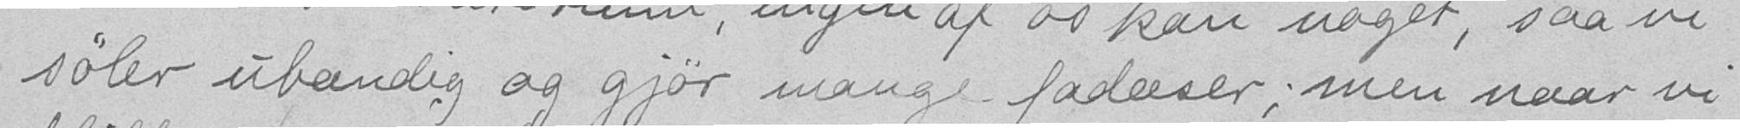søler ubændig og gjør mange fadæser; men naar vi
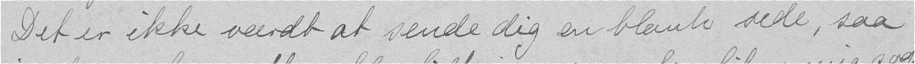Det er ikke værdt at sende dig en blank side, saa
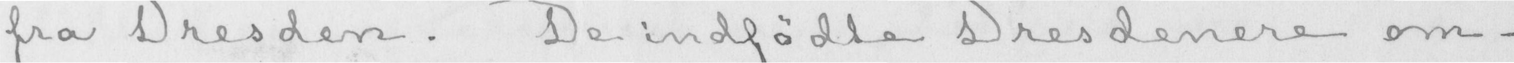fra Dresden. De indfødte Dresdenere om-
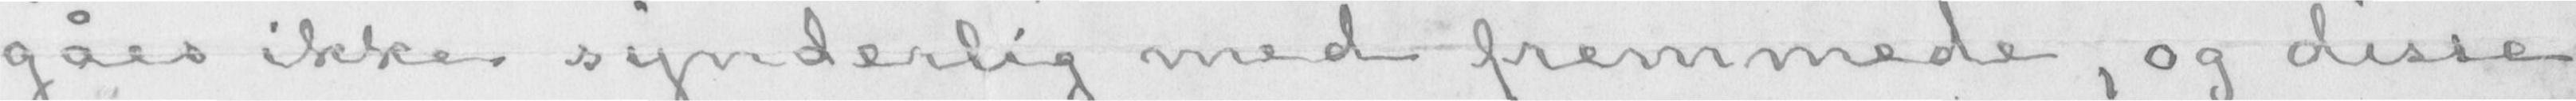gåes ikke synderlig med fremmede, og disse
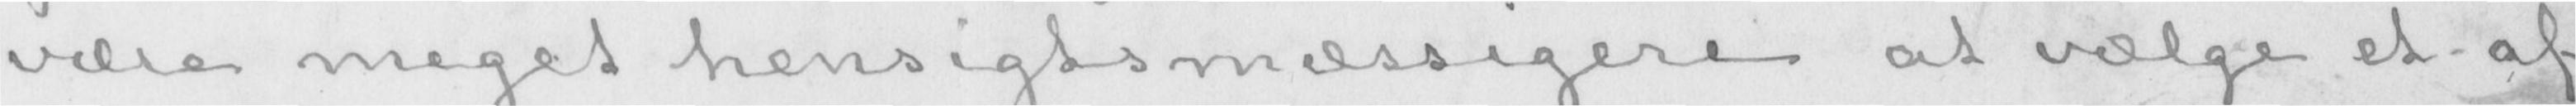være meget hensigtsmæssigere at vælge et af
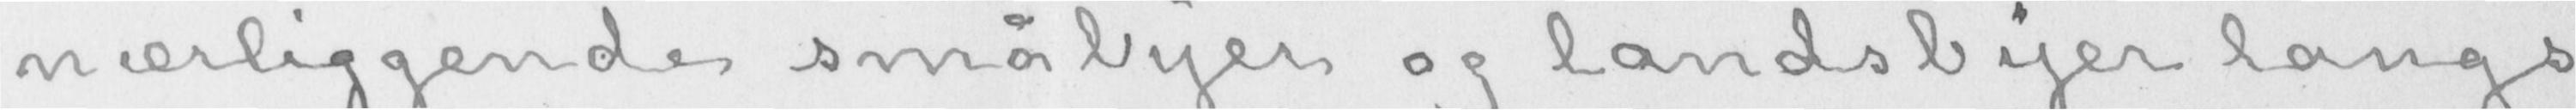nærliggende småbyer og landsbyer langs
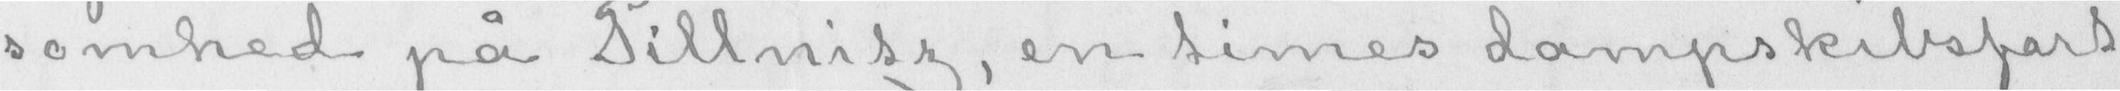somhed på Pillnitz, en times dampskibsfart
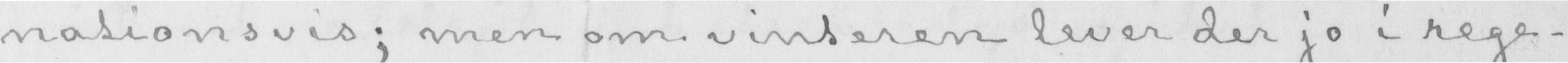nationsvis; men om vinteren lever der jo i rege-
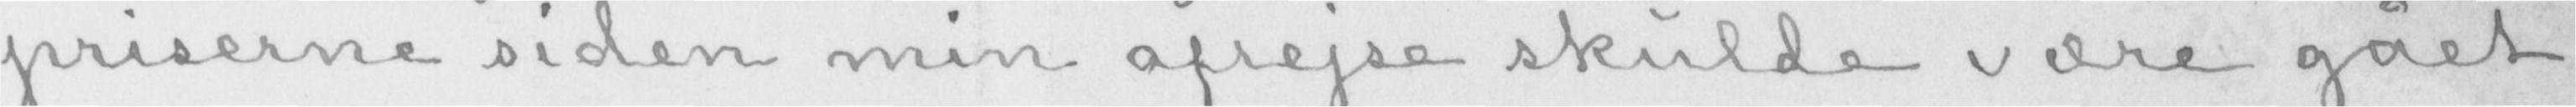priserne siden min afrejse skulde være gået
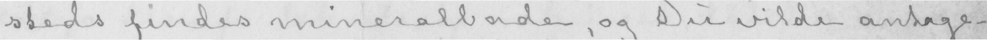steds findes mineralbade, og Du vilde antage-
